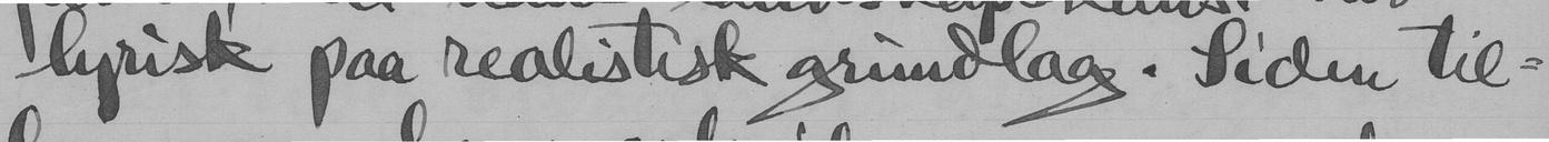lyrisk paa realistisk grundlag. Siden til-
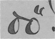dø.
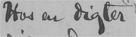Hos en digter
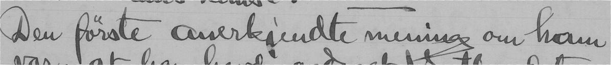Den første anerkjendte mening om ham
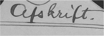afskrift.
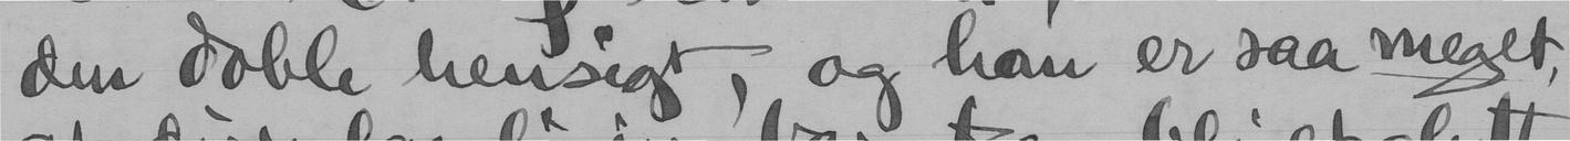den doble hensigt, og han er saa meget,
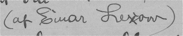(af Einar Lexow)
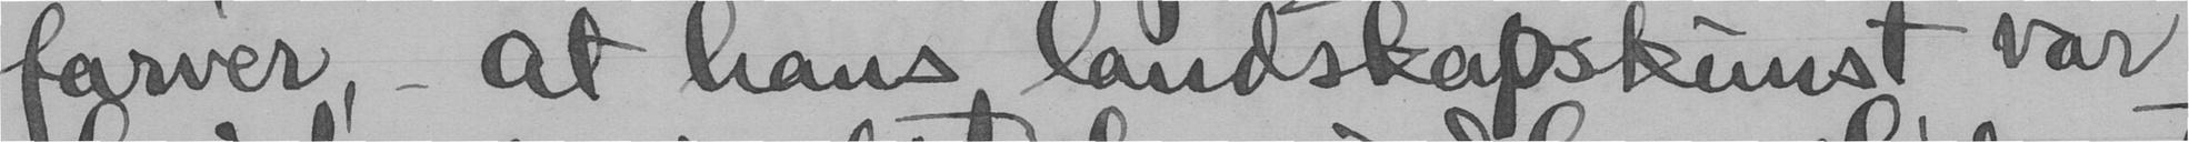farver, - at hans landskabskunst var
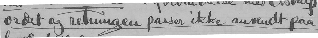ordet og retningen passer ikke anvendt paa
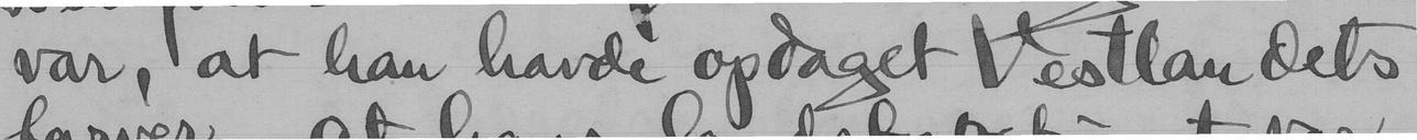var, at han havde opdaget Vestlandets
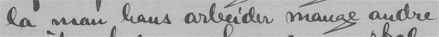la man hans arbeider mange andre
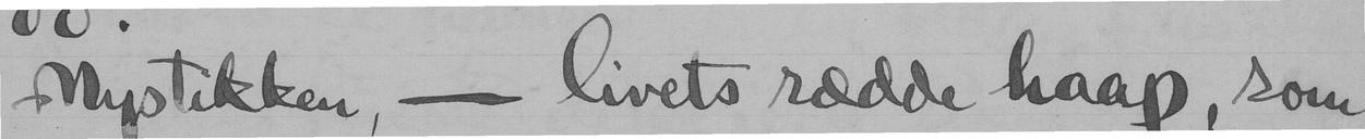Mystikken, - livets rædde haap, som
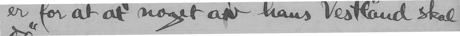er "for at at noget av hans Vestland skal
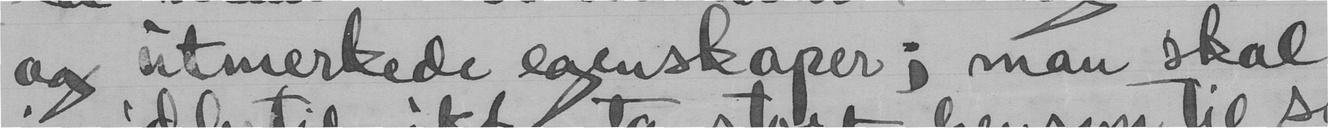og utmerkede egenskaper; man skal
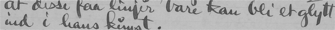at disse faa linjer bare kan bli et glytt
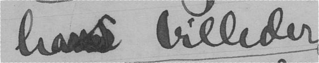hans billeder.
